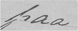paa
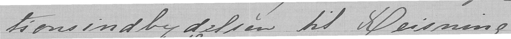tionsindbydelsen til Reisning,
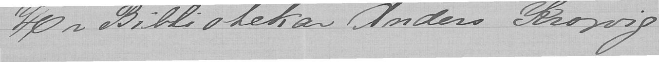Hr Bibliotekar Anders Krogvig
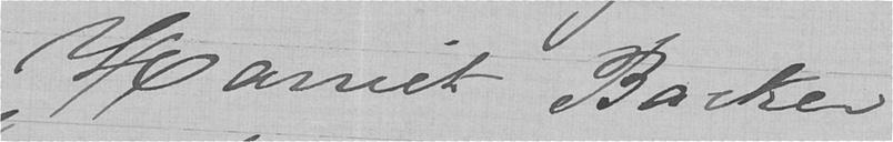Harriet Backer
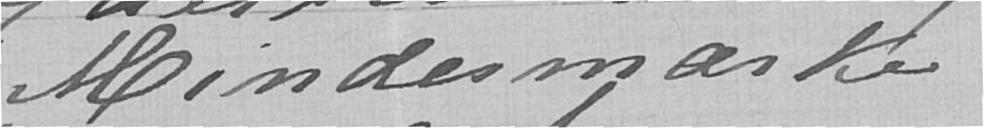Mindesmærke
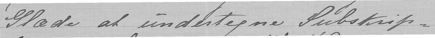Glæde at undertegne Subskrip-
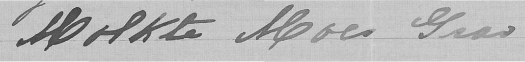Molkte Moes Grav
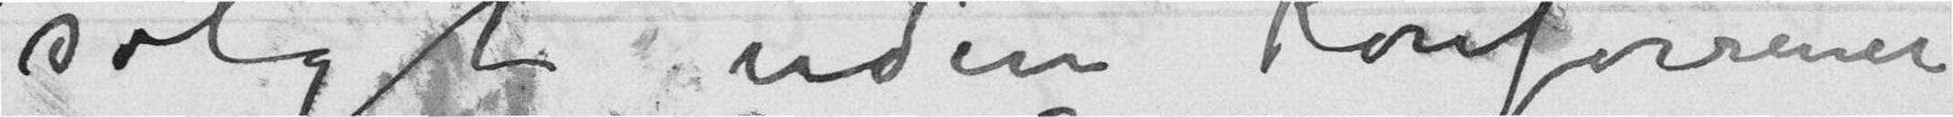solgt uden Konference
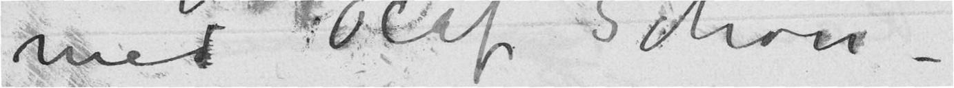med Olaf Schou –
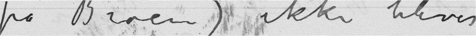på Broen) ikke bliver
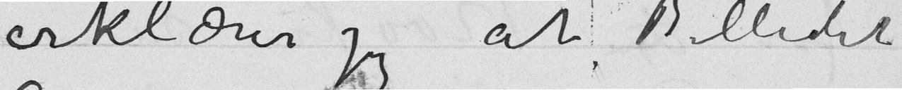erklærer jeg at Billedet
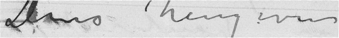Deres hengivne
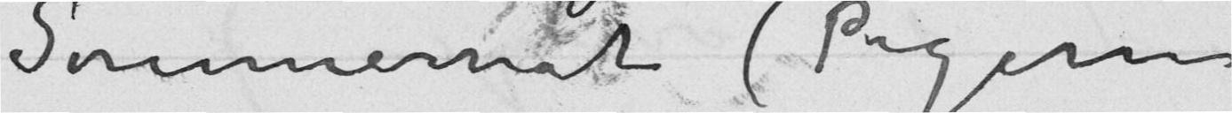Sommernat (Pigerne
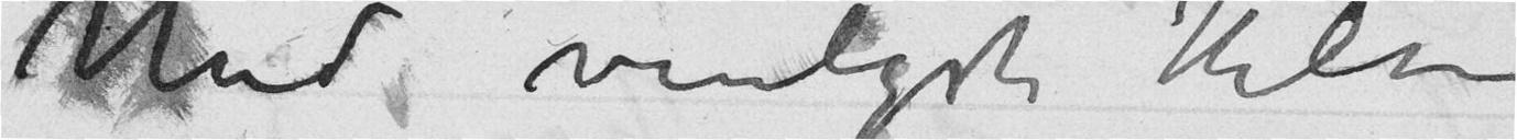Med venligste Hilsen
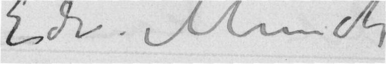Edv. Munch
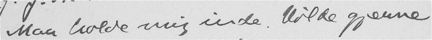Maa holde mig inde. Vilde gjerne
